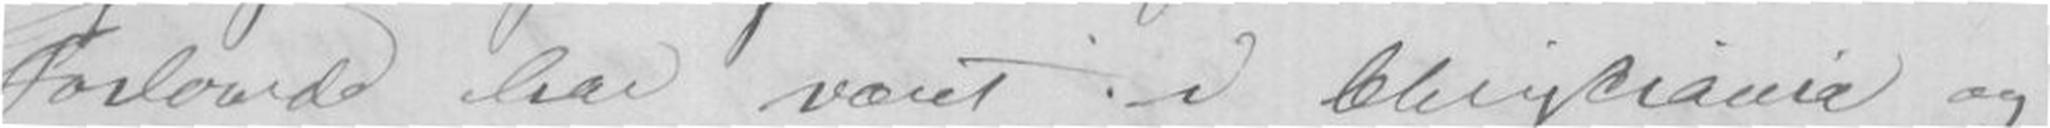Forlovede har været i Christiania og
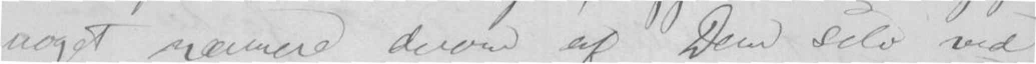noget nærmere derom af Dem selv ved
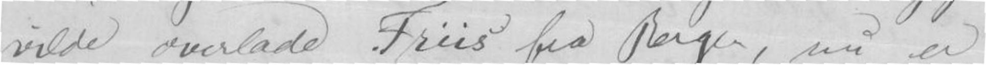vilde overlade Friis fra Bergen, nu er
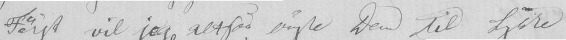Først vil jeg altsaa ønske dem til Lykke
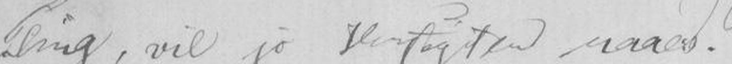Ting, vil jo Hensigten naaes.
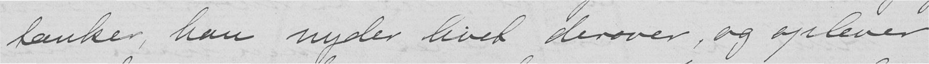tænker, han nyder livet derover, og oplever
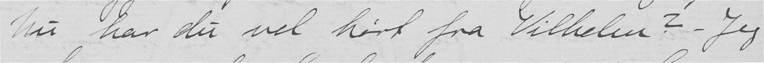Nu har du vel hørt fra Vilhelm? – Jeg
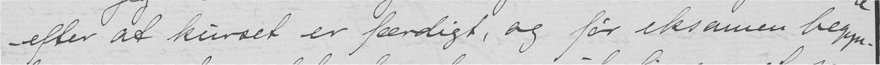– efter at kurset er færdigt, og før eksamen begyn-
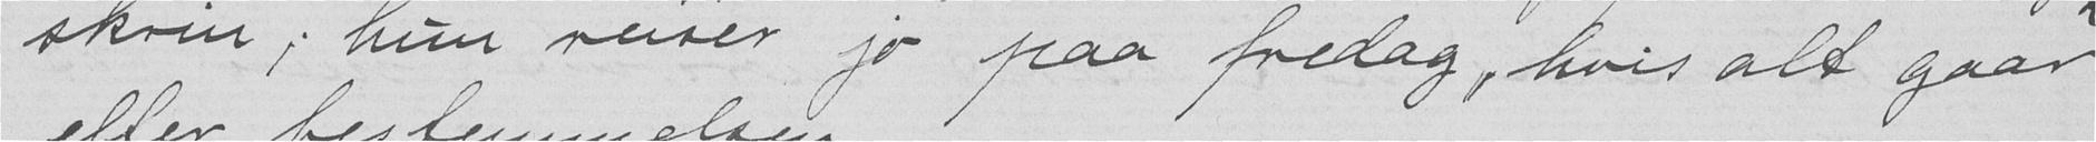skrin; hun reiser jo paa fredag, hvis alt gaar
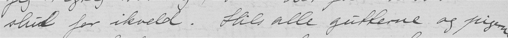slut for ikveld. Hils alle gutterne og pigerne
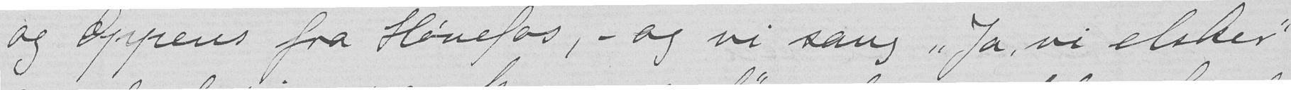og Oppens fra Hønefos, – og vi sang "Ja, vi elsker"
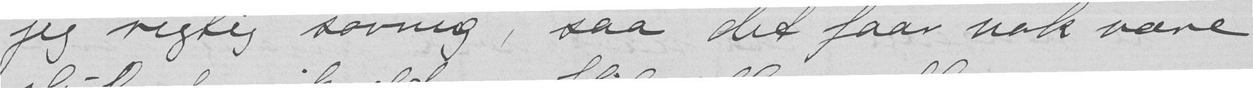jeg rigtig søvnig, saa det faar nok være
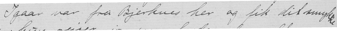I gaar var fru Bjerknes her og fik dit smykke-
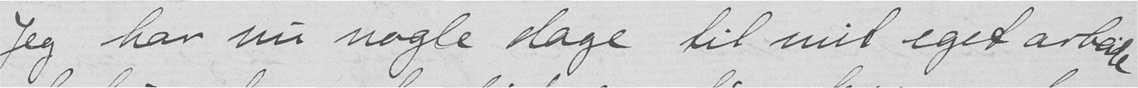Jeg har nu nogle dage til mit eget arbeide
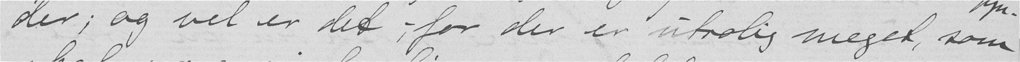der; og vel er det, – for der er utrolig meget, som
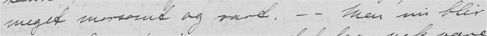meget morsomt og rart. – – Men nu blir
